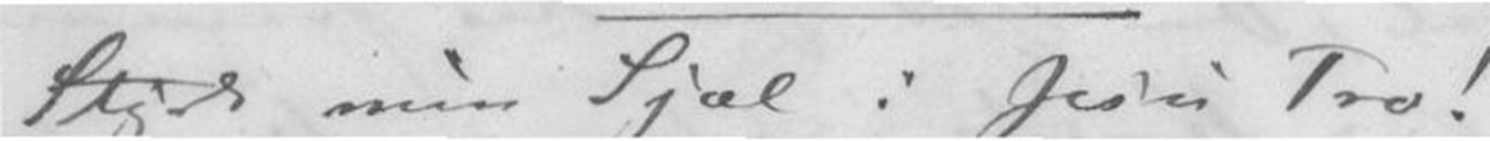Styrk min Sjæl i Jesu Tro!
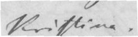Kristina.
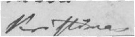Kristina.
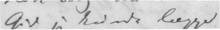Gid jeg kunde lægge
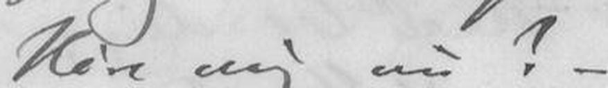Høre mig nu? -
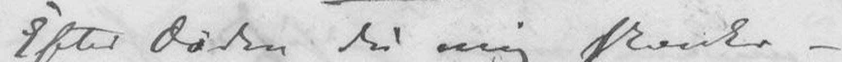Efter Døden du mig skænker -
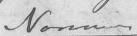Nonnerne.
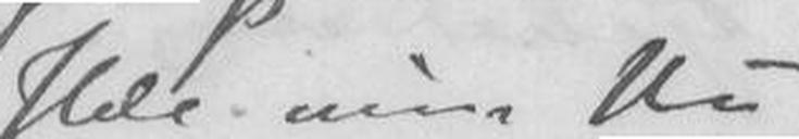Hele min Hu
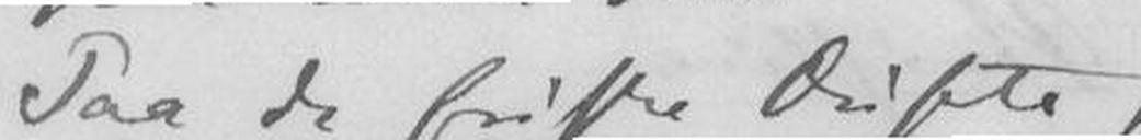Paa de friske Dufte,
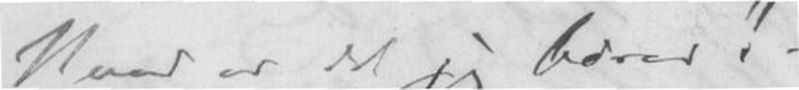Hvad er det jeg hører?
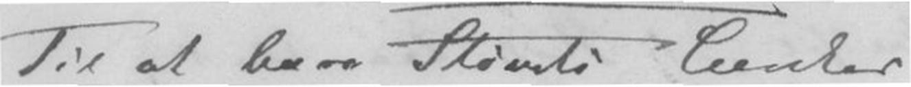Tid at have Støvets Lænker -
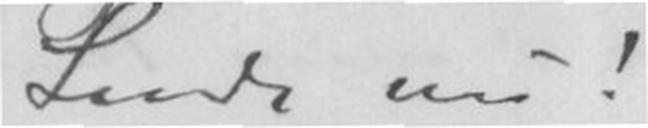Sende nu!
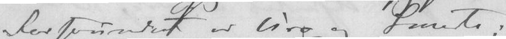Forsvundet er Uro og Smerte;
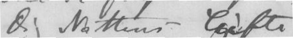Dig Nattens Lufte
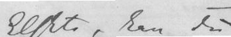Elskte,kan du
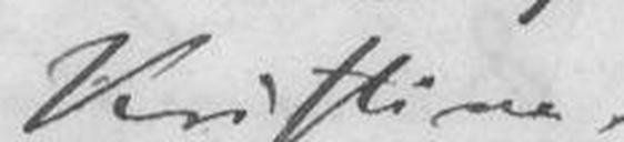Kristina.
